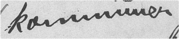kommuner.
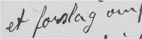et forslag om
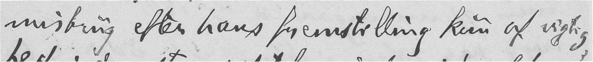misbrug efter hans fremstilling kun af vigtig-
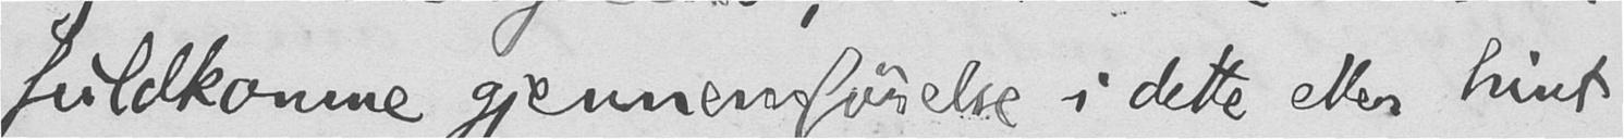fuldkomne gjennemførelse i dette eller hint
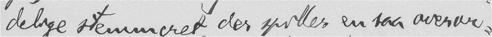delige stemmeret, der spiller en saa overor-
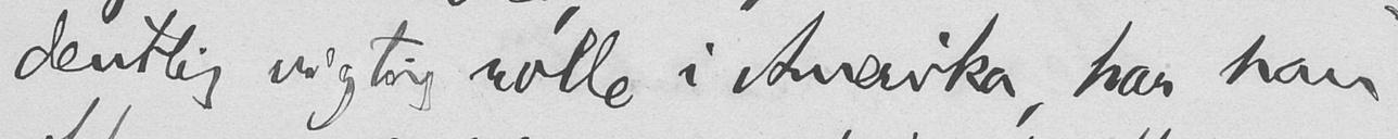dentlig vigtig rolle i Amerika, har han
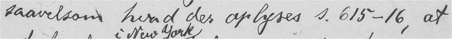saavelsom hvad der oplyses s. 615-16, at
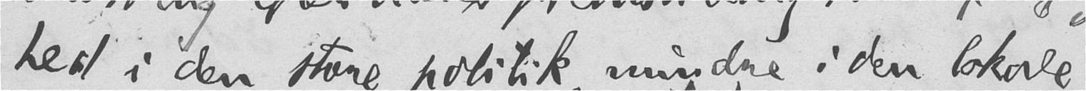hed i den store politik, mindre i den lokale,
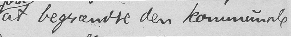at begrændse den kommunale
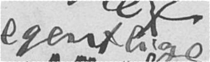egentlige
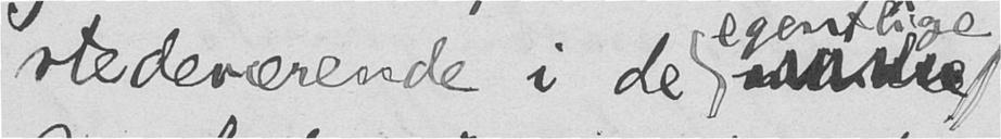stedeværende i de
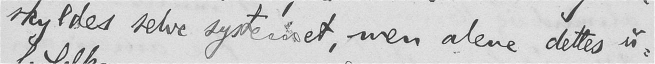skyldes selve systemet, men alene dettes u-
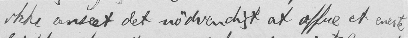ikke anseet det nødvendigt at offre et eneste
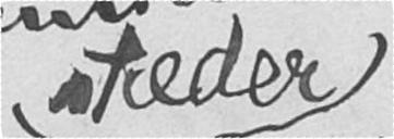steder
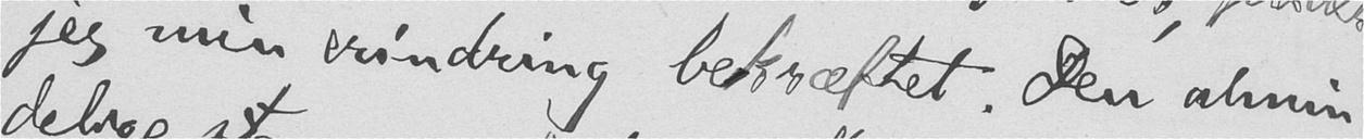jeg min erindring bekræftet. Den almin-
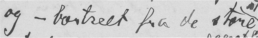og – bortseet fra de store
